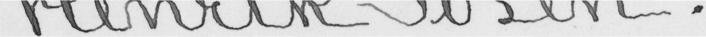Henrik Ibsen.
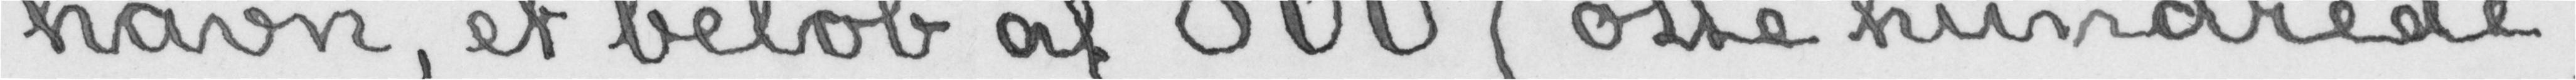havn, et beløb af 800 (otte hundrede)
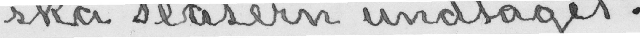ska Teatern undtaget.
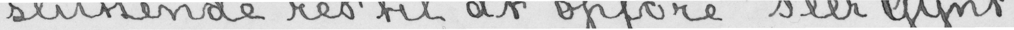sluttende ret til dat opføre «Peer Gynt»
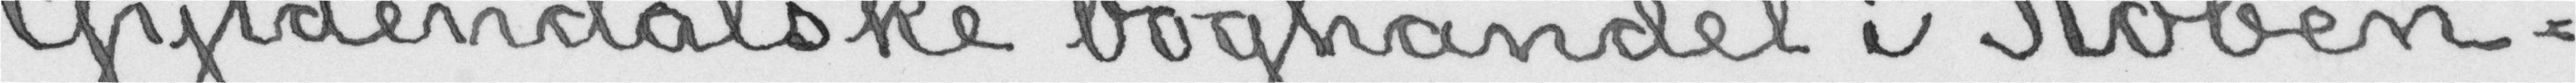Gyldendalske boghandel i Køben-
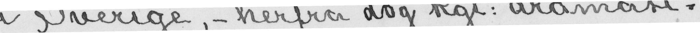i Sverige,- herfra dog kgl: dramati-
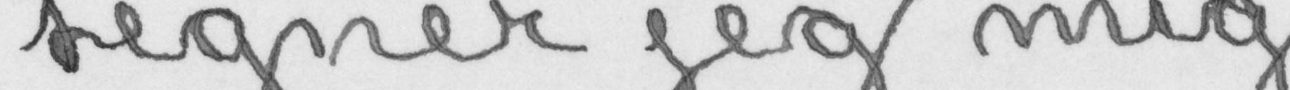tegner jeg mig
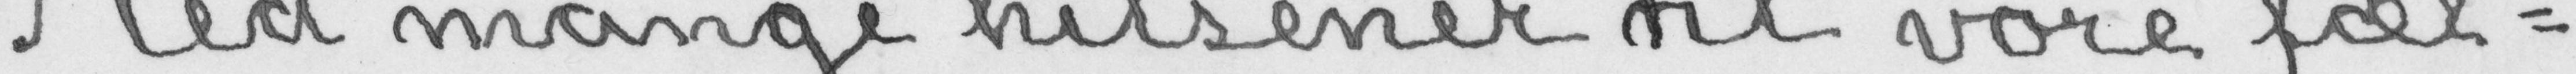Med mange hilsener til vore fæl-
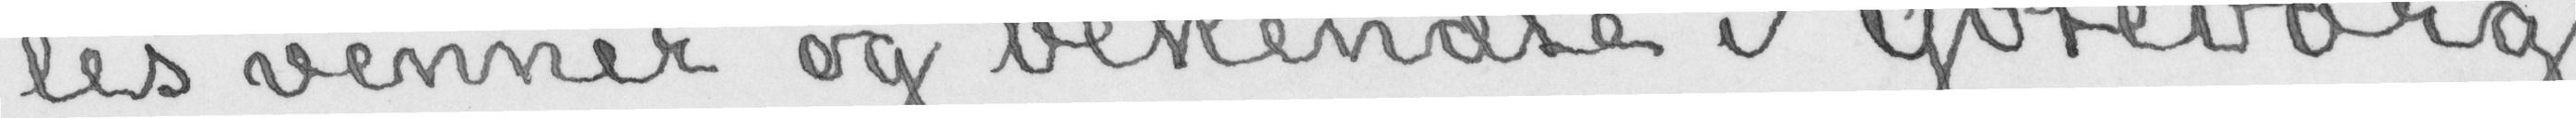les venner og bekendte i Gøteborg
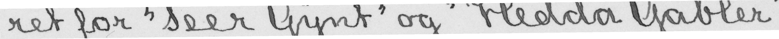ret for «Peer Gynt» og «Hedda Gabler»,
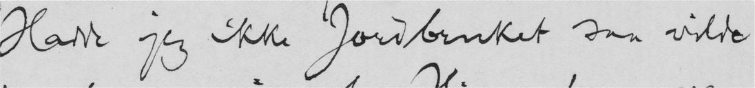Hadde jeg ikke Jordbruket saa vilde
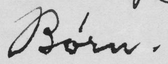Børn.
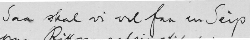Saa skal vi vel faa en Seip
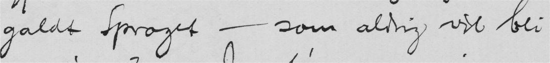galdt Sproget - som aldrig vil bli
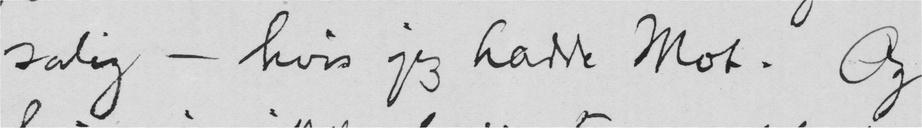salig - hvis jeg hadde Mot. Og
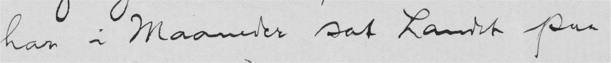har i Maaneder sat Landet paa
